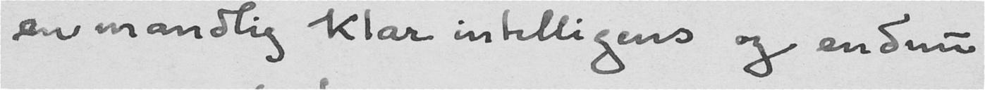en mandlig klar intelligens og endnu
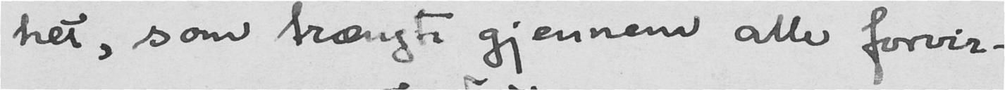het, som trængte gjennem alle forvir-
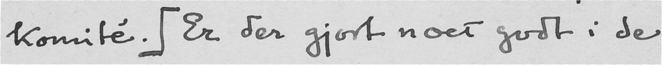komité. Er der gjort noet godt i de
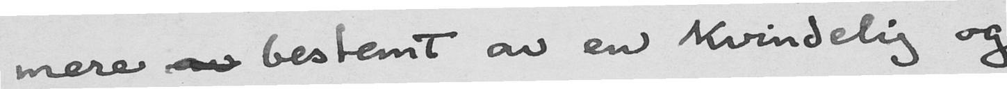mere av bestemt av en kvindelig og
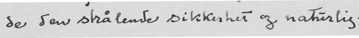de den strålende sikkerhet og naturlig-
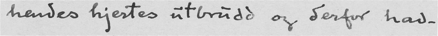hendes hjertes utbrudd og derfor had-
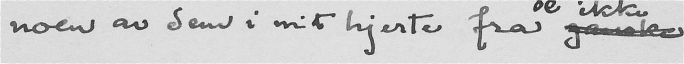noen av dem i mit hjerte fra ganske
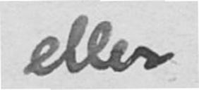eller
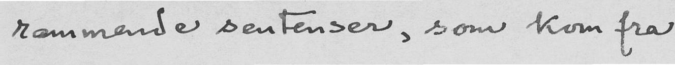rammende sentenser, som kom fra
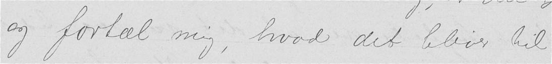og fortæl mig, hvad det bliver til
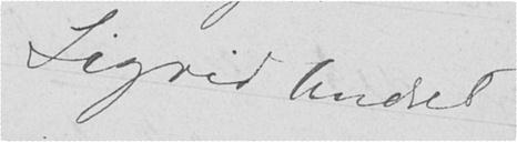Sigrid Undset
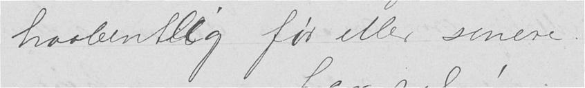haabentlig før eller senere.
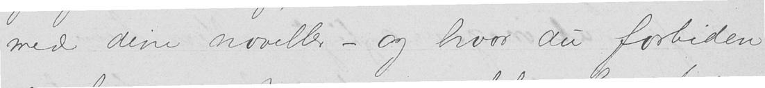med dine noveller - og hvor du fortiden
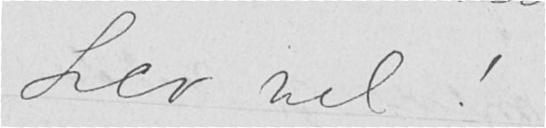Lev vel!
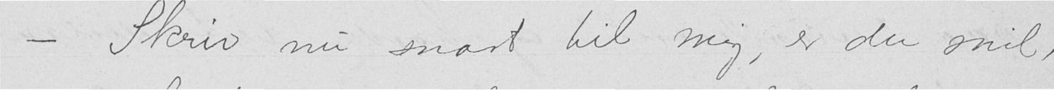- Skriv nu snart til mig, er du snil,
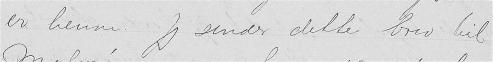er henne. Jeg sender dette brev til
